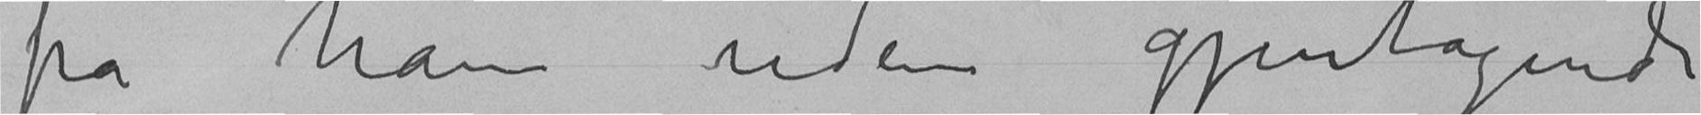fra ham uden gjentagende
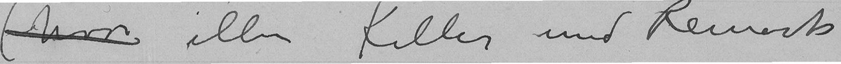(hvor eller Keller und Reinerts
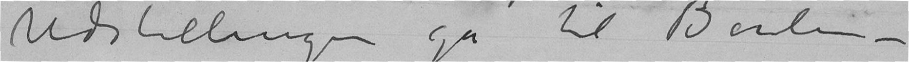Udstillingen gå til Berlin –
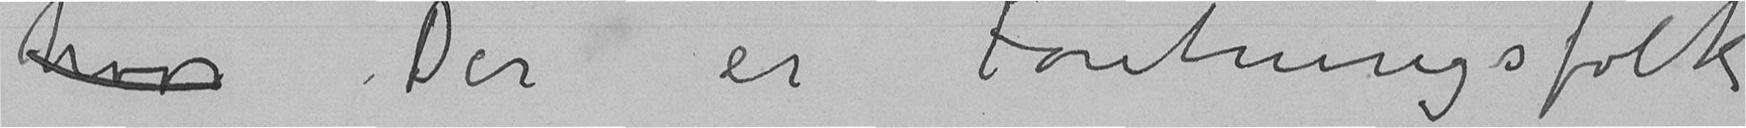hvor Der er Foretningsfolk
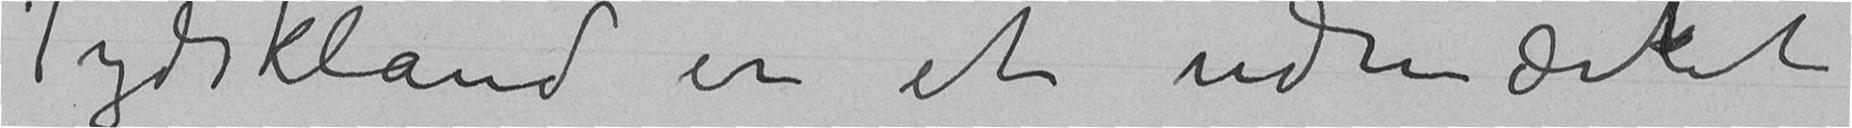Tydskland er et udmærket
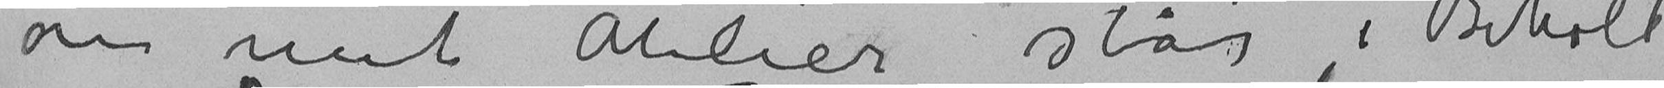om mit Atelier står i Behold
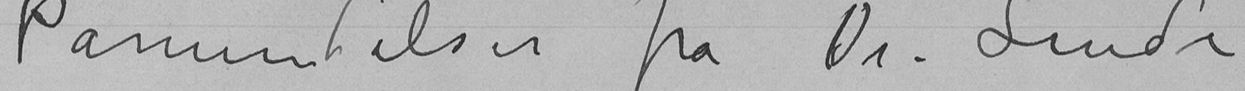Påmindelser fra Dr. Linde
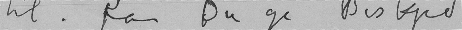til. Kan Du gi Beskjed
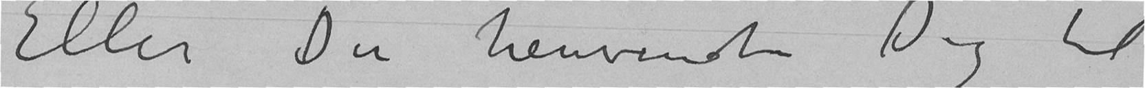Eller Du henvendte Dig til
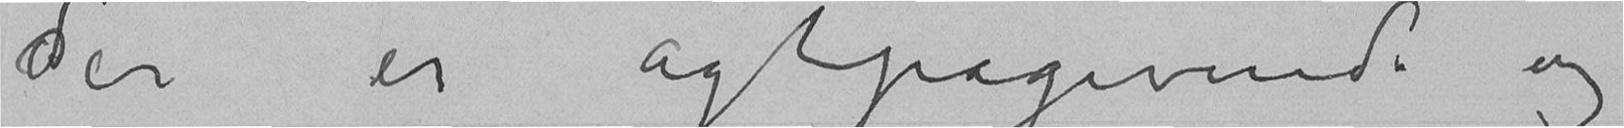der er agtpågivende og
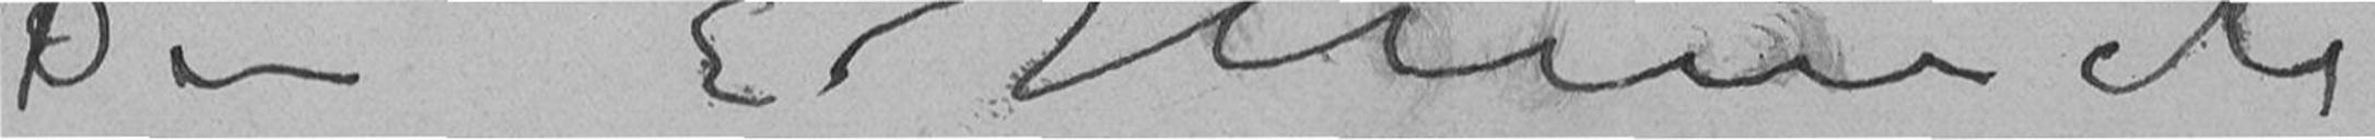Din E Munch
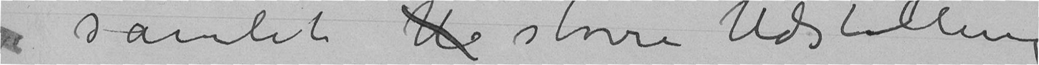samlet U større Udstilling
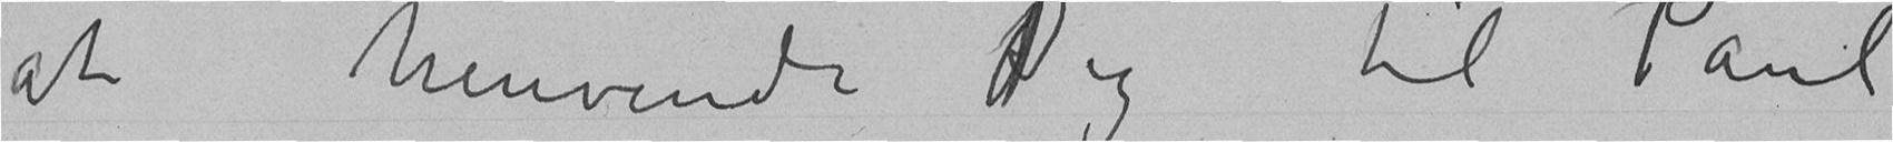at henvende Dig til Paul
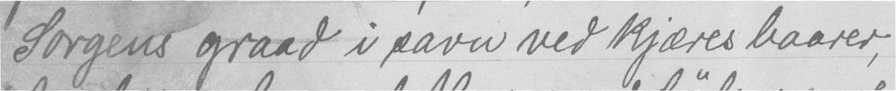Sorgens graad i savn ved kjæres baarer,
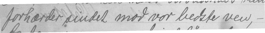forhærder sindet mod vor bedste ven, -
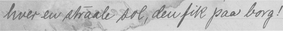hver en straale sol, den fik paa borg!
